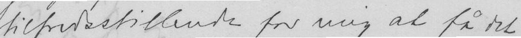tilfredsstillende for mig at få det
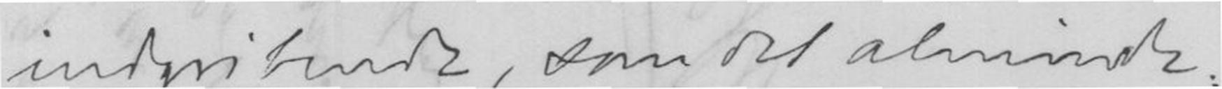indgribende, som det alminde
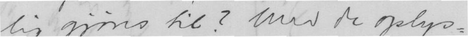lig gjøres til? Med de oplys-
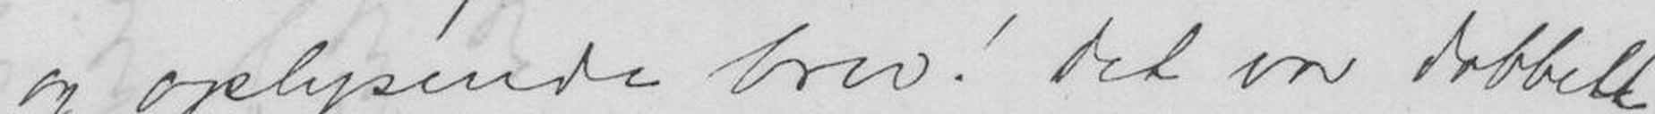og oplysende brev!. Det var dobbelt
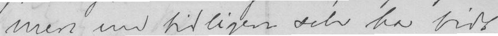mere end tidligere selv har bidt
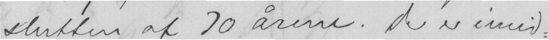slutten af 20 årene. Der er imid-
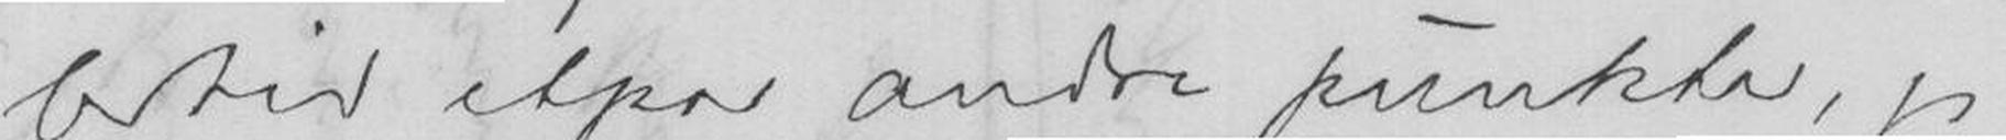lertid etpar andre punkter, jeg
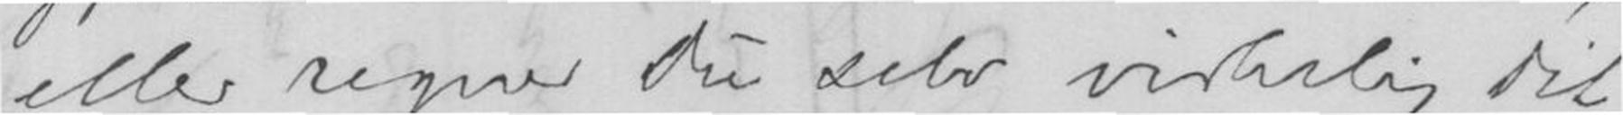eller regner du selv virkelig dit
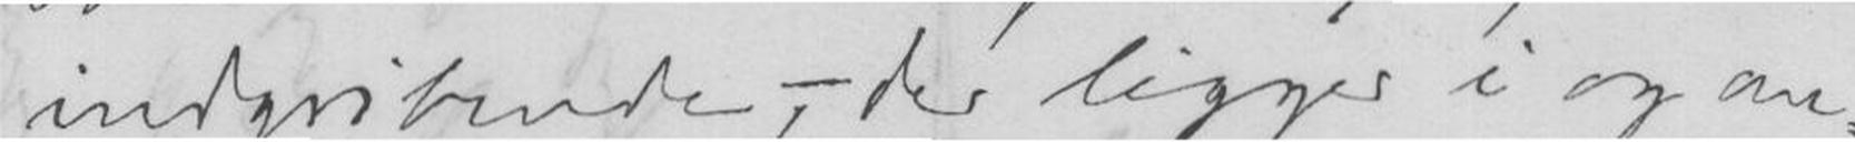indgribende,- der ligger i og om-
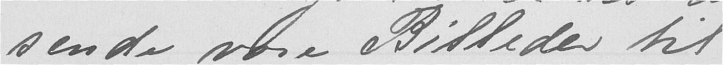sende vore Billeder til
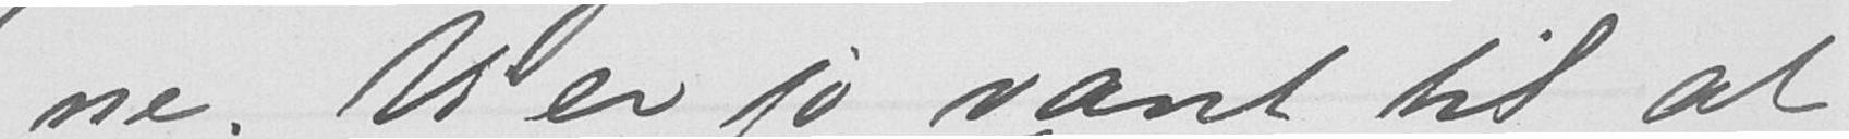ne. Vi er jo vant til at
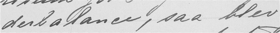derbalance, saa blev
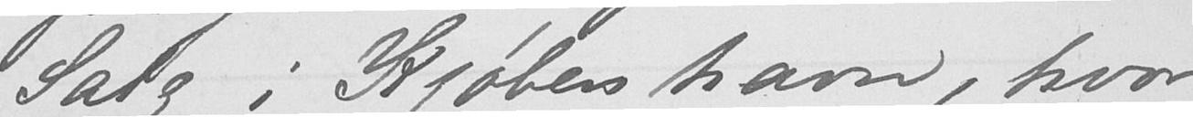Salg i Kjøbenhavn, hvor
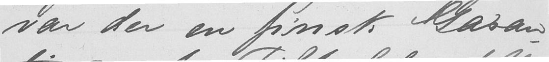var der en finsk Garan-
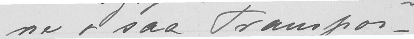ne, saa Transpor-
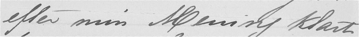efter min Mening klart
